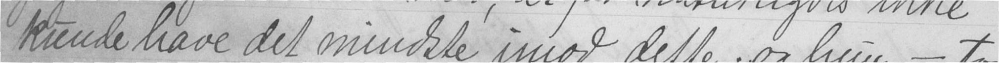kunde have det mindste imod dette; og hun - tog
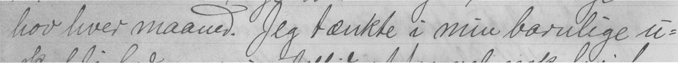hov hver maaned. Jeg tænkte i min barnlige u-
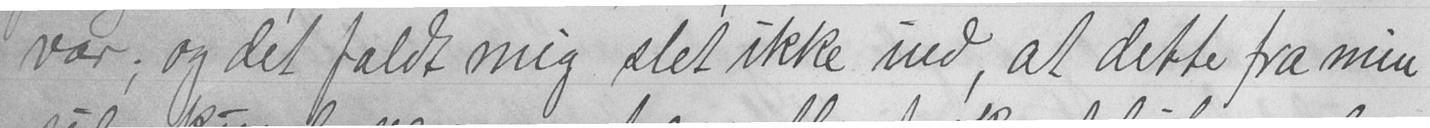var; og det faldt mig slet ikke ind, at dette fra min
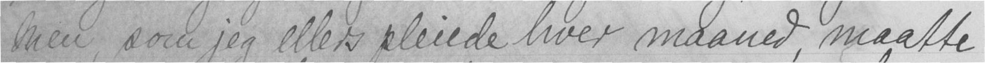Men, som jeg ellers pleiede hver maaned, maatte
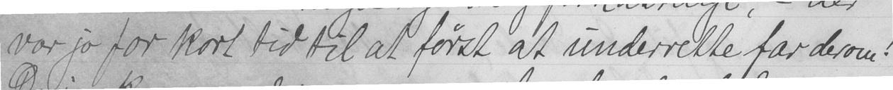var jo for kort tid til at først at underrette far derom!
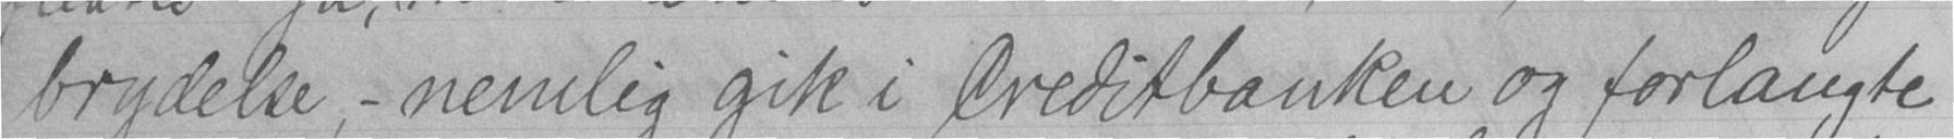brydelse, - nemlig gik i Creditbanken og forlangte
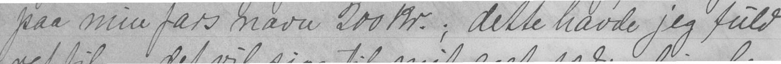paa min fars navn 200 kr.; dette havde jeg fuld
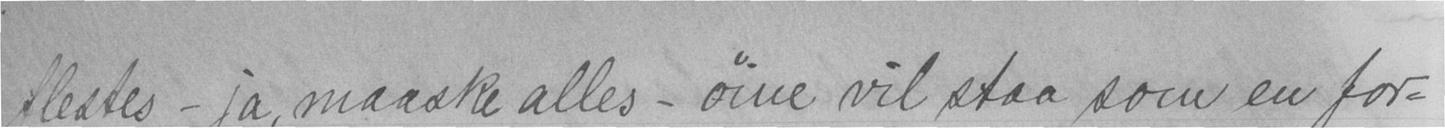flestes - ja, maaske alles - øine vil staa som en for-
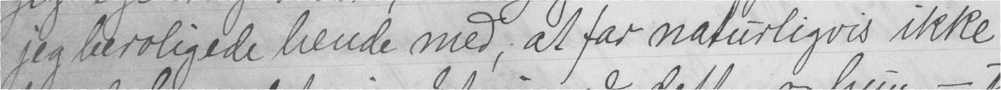jeg beroligede hende med, at far naturligvis ikke
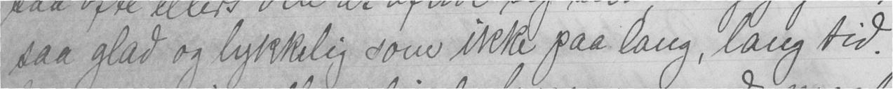saa glad og lykkelig som ikke paa lang, lang tid.
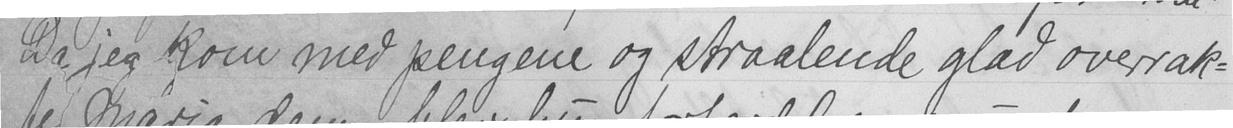Da jeg kom med pengene og straalende glad overrak-
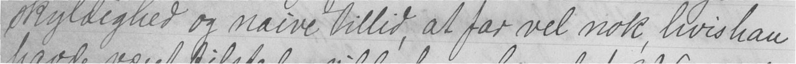skyldighed og naive tillid, at far vel nok, hvis han
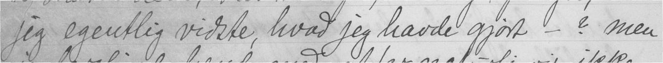jeg egentlig vidste, hvad jeg havde gjort - ? men
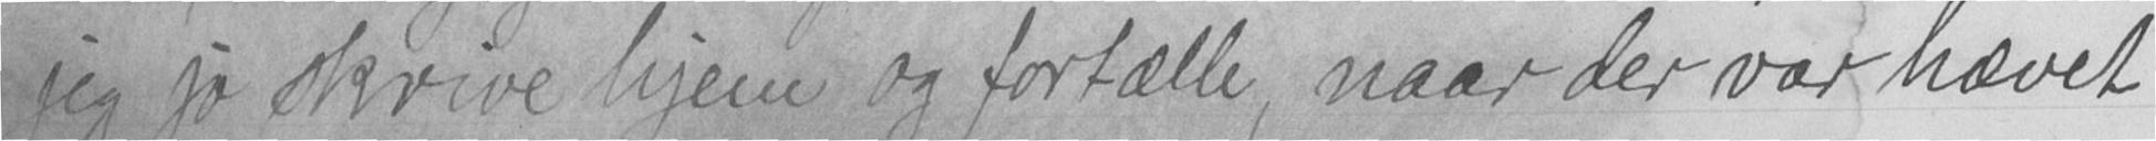jeg jo skrive hjem og fortælle, naar der var hævet
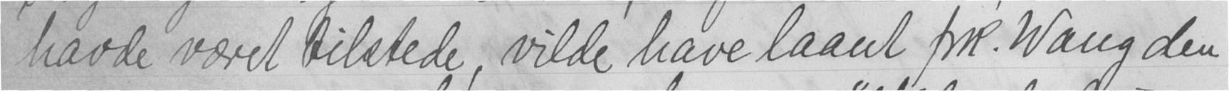havde været tilstede, vilde have laant frk. Wang den
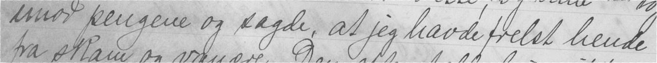imod pengene og sagde, at jeg havde frelst hende
